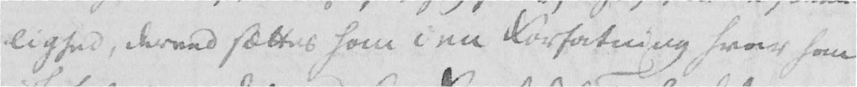lighed, dermed sættes han i en Forfatning hvor han
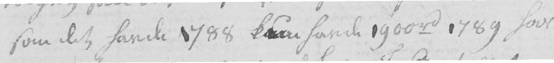som det havde 1788 kun havde 1900 rd 1789 har
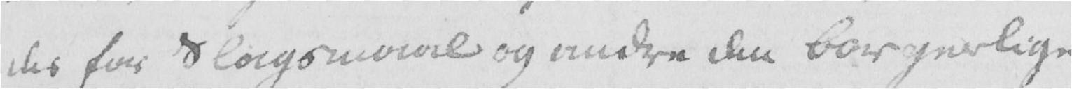des for Slagsmaal og andre den borgerlige
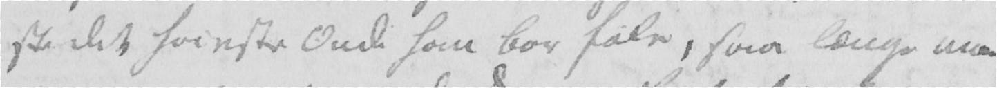ste det høieste Onde han bør føle, saa længe man
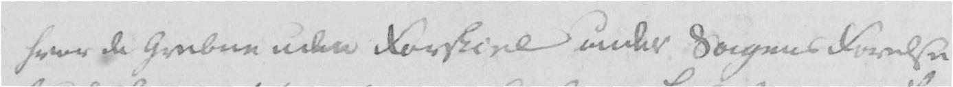hvor de Grebne uden Forskiel under Sagens Førelse
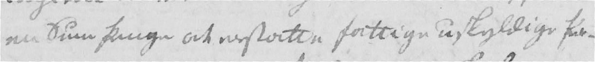en Sum Penge at erstatte fattige uskyldige Per-
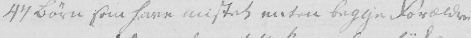47 Børn som have mistet enten begge Forældre
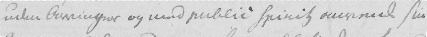uden Arvinger og med public spirit omvende sin
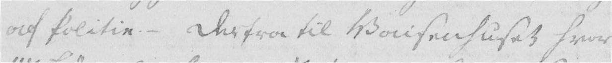af Politie. - Derfra til Waisenhuset hvor
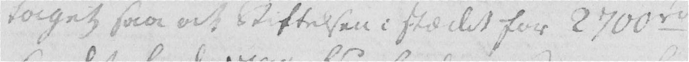taget saa at Stiftelsen i stædet for 2700 rd
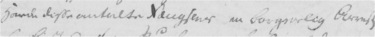Havde disse omtalte Fængsler en borgerlig Arrest
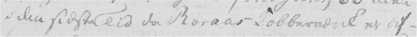i den sidste Tid da Røraas Kobberværck er af-
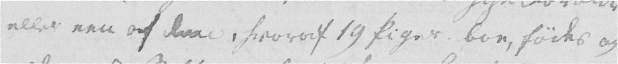eller een af dem, hvoraf 19 Piger boe, fødes og
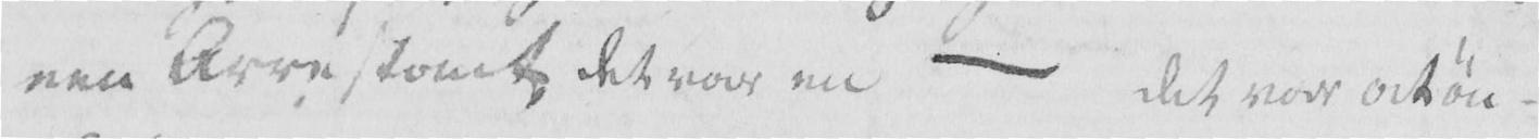een Arrestant det vare en - det var at øn-
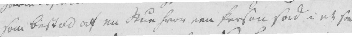som bestod af en Stue hvor een Person sad i et saa-
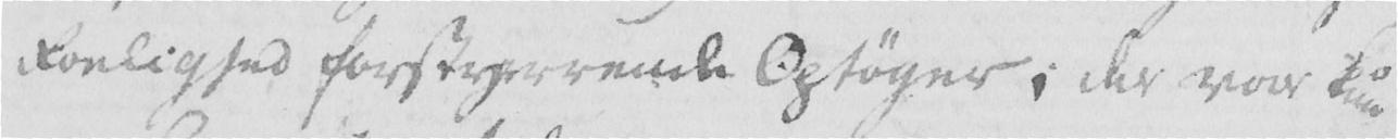Roelighed forstyrrende Optøyer; der var kun
